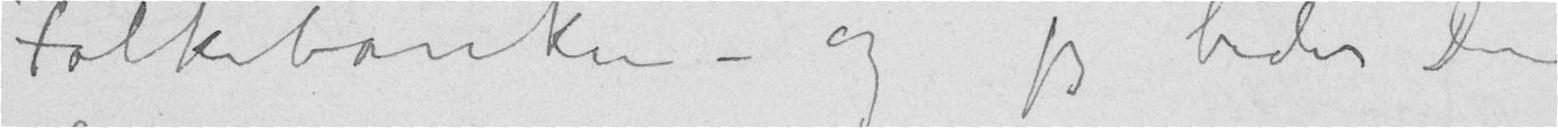Folkebanken – og jeg beder Dem
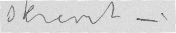skrevet –
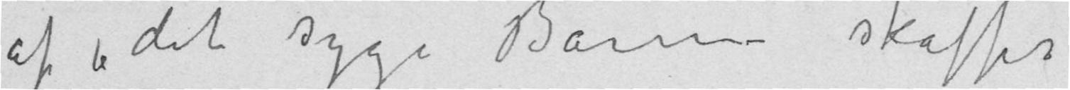af «det syge Barn» skaffer
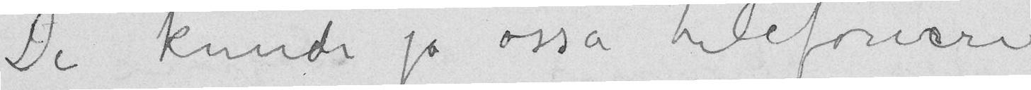De kunde jo osså telefonere
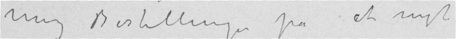mig Bestilling på et nyt
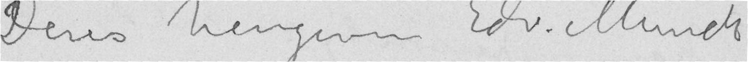Deres hengivne Edv. Munch
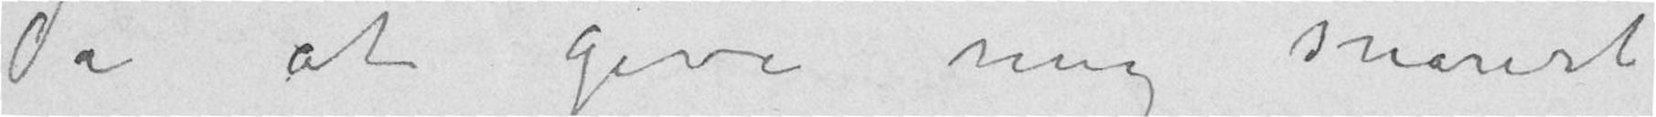da at give mig snarest
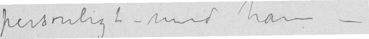personligt med ham –
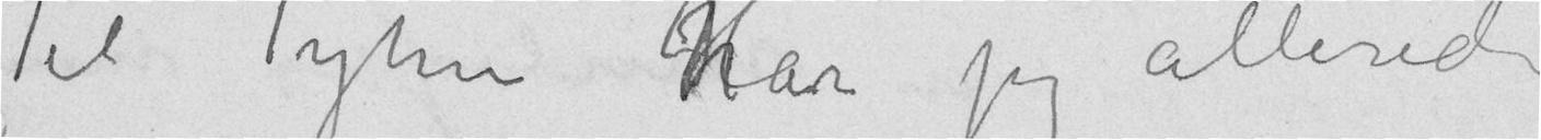Til Fyhn har jeg allerede
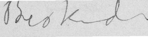Besked –
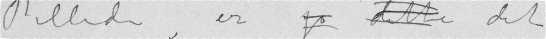Billede, er jo dette det
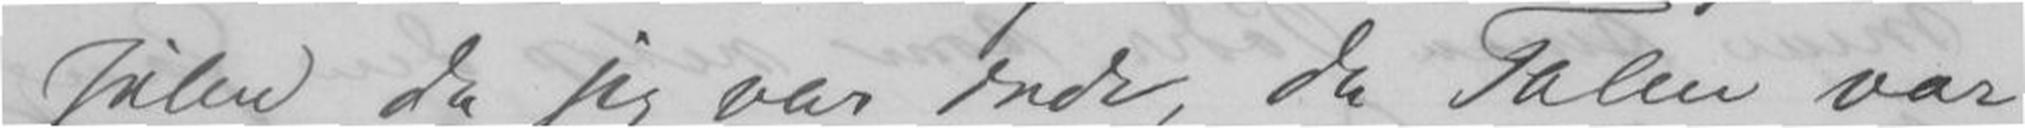Julen da jeg var inde, da Talen var
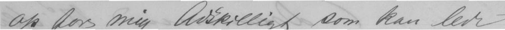op for mig Adskilligt som kan lede
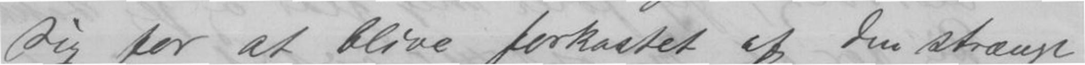sig for at blive forkastet af den strænge
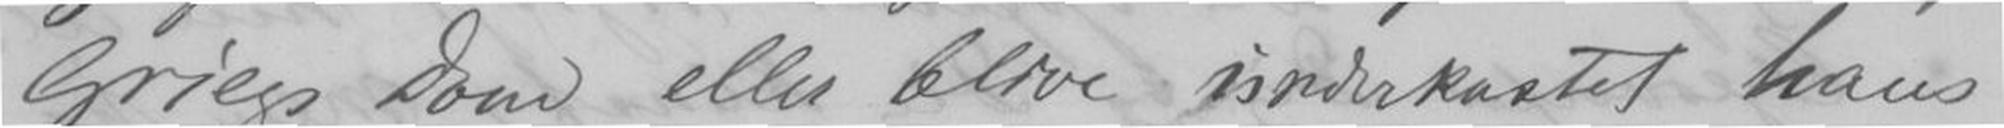Griegs Dom eller blive underkastet hans
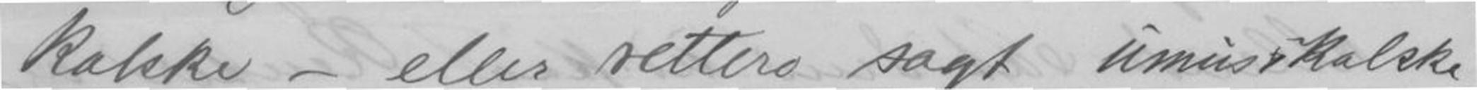eller rettere sagt umusikalske
